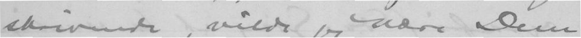skrivende, vilde jeg være Dem
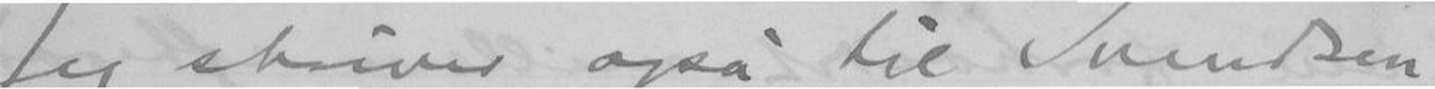Jeg skriver også til Svendsen
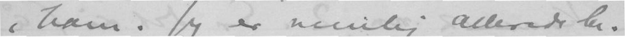i ham. Jeg er nemlig allerede be-
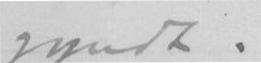gyndt.
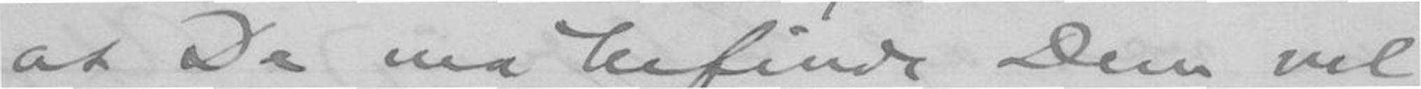at De må befinde Dem vel
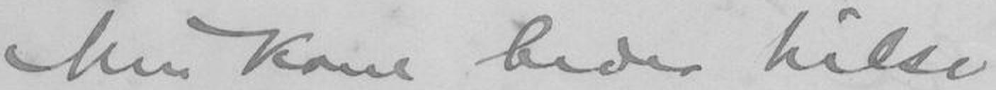Min kone beder hilse
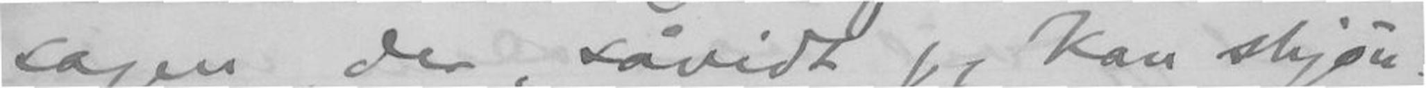sagen, der, såvidt jeg kan skjøn-
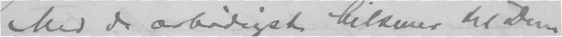Med de ærbødigste hilsener til Dem
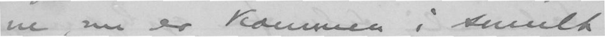ne, nu er kommen i smult
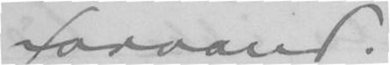farvand.
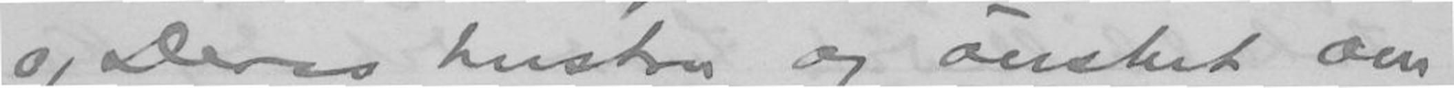og Deres hustru og ønsket om,
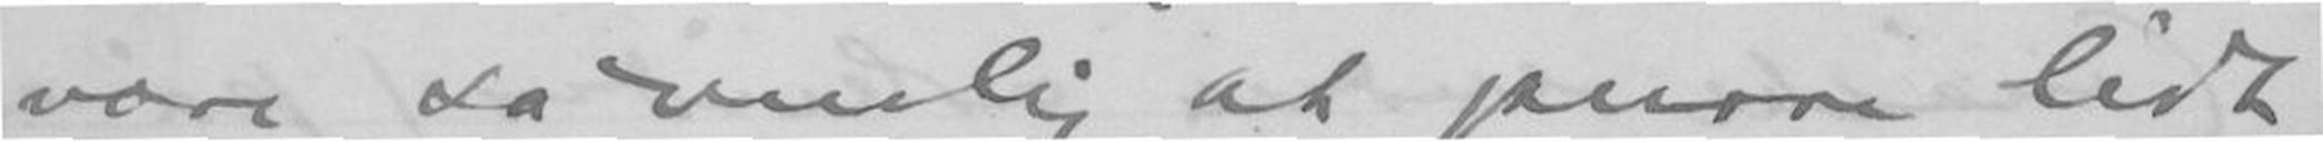være såvenlig at purre lidt
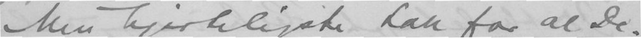Men hjerteligste tak for al De-
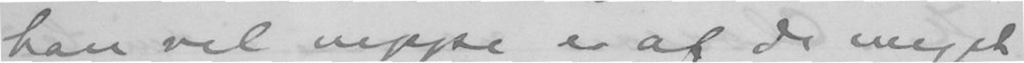han vel neppe er af de meget
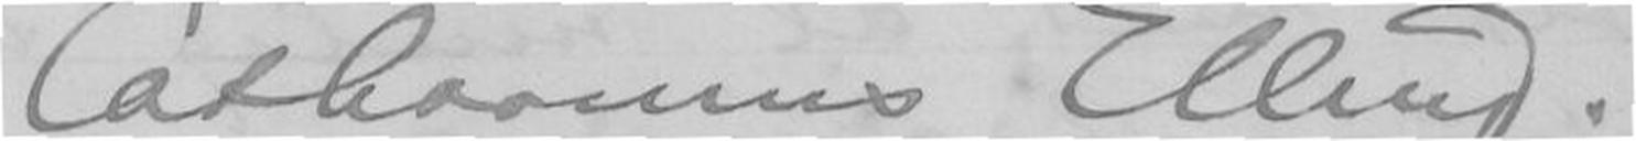Catharinus Elling.
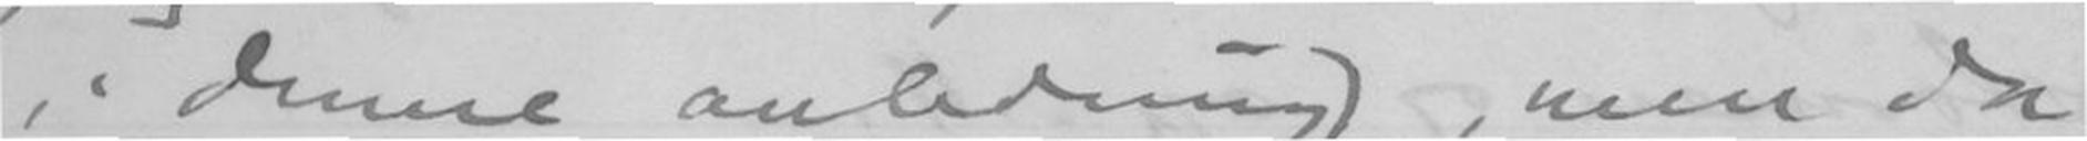i denne andledning, men da
